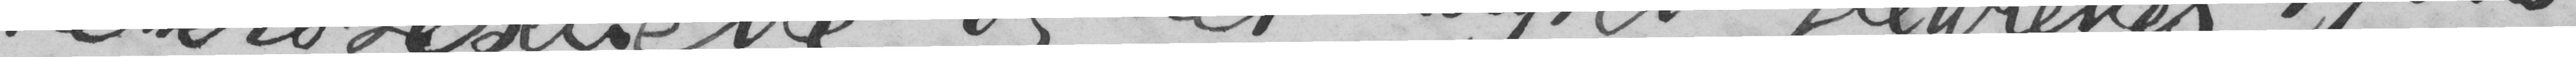heterosexuelle, og det uagtet fedrenes kjøns-
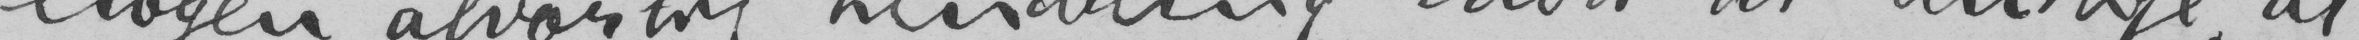nogen alvorlig hindring mot at antage, at
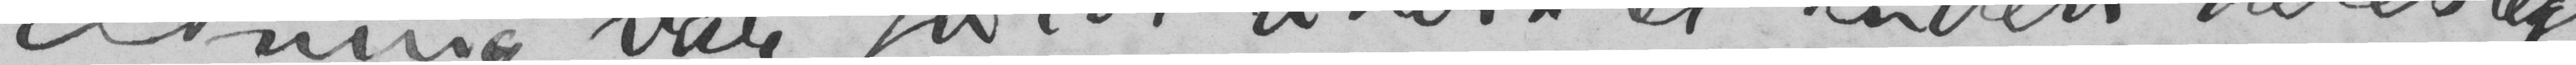retning var fuldt udviklet inden deres ægte-
